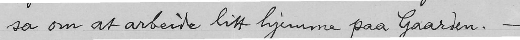sa om at arbeide litt hjemme paa Gaarden. -
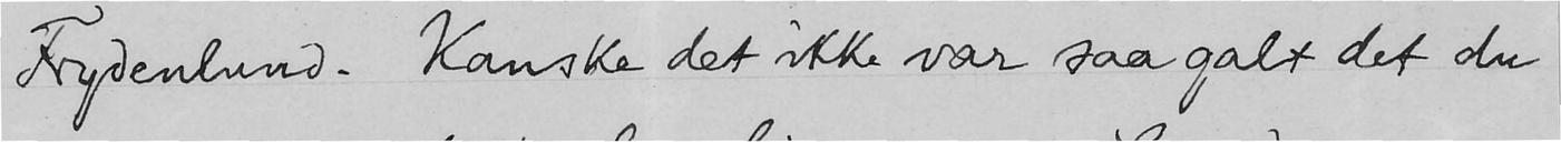Frydenlund. Kanske det ikke var saa galt det du
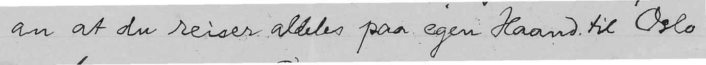an at du reiser aldeles paa egen Haand til Oslo
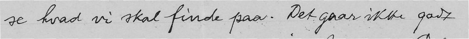se hvad vi skal finde paa. Det gaar ikke godt
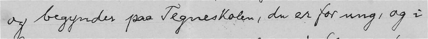og begynder paa Tegneskolen, du er for ung, og i
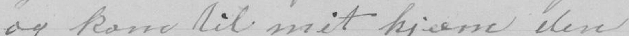og kom til mit hjæm den
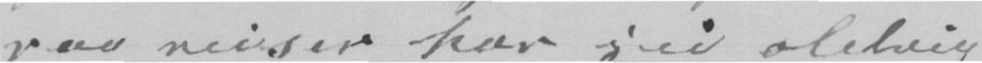paa reiser har jei aldrig
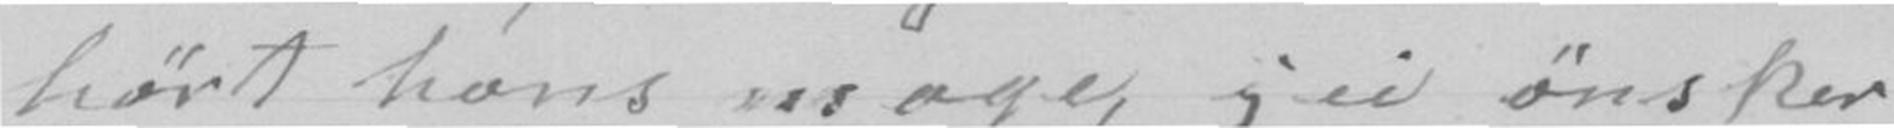hørt hans mage, jei ønsker
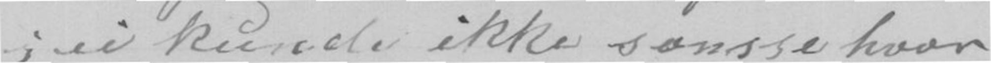jei kunde ikke sansse hvor
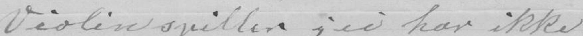Violinspiller jei har ikke
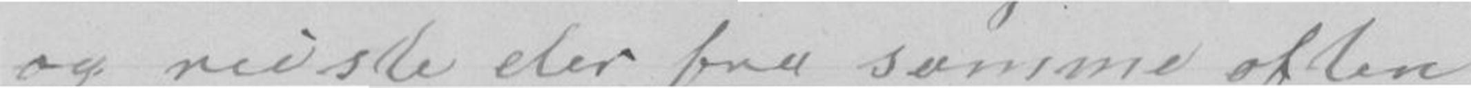og reiste der fra samme aften
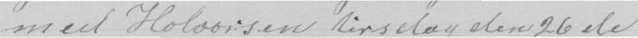med Holvorsen tirsdag den 26de
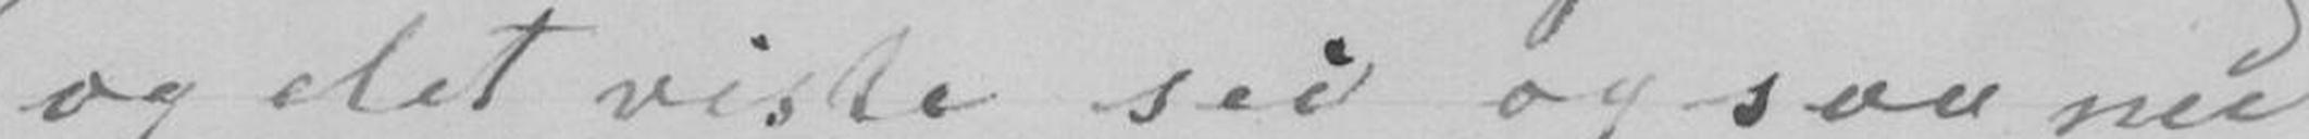og det viste sei ogsaa nu
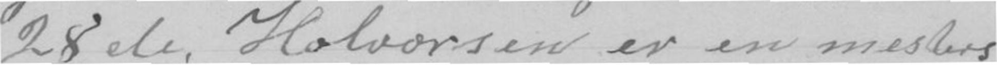28de. Holvorsen er en mesters
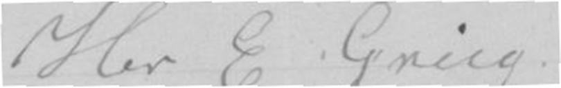Her E. Grieg.
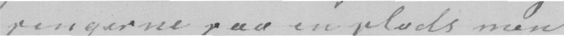pengerne paa en plads men
